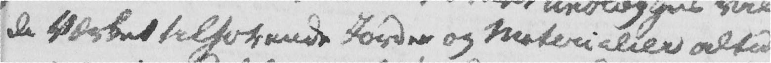de Værket tilhørende Jorder og Materialier altid
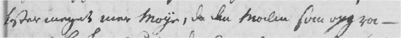koster meget mer Møye, da den Malm som opgra-
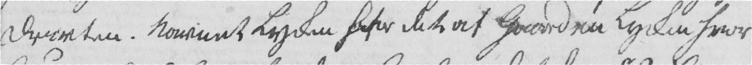Drivten. Navnet Lycken haver det af Gaarden Lycken hvor
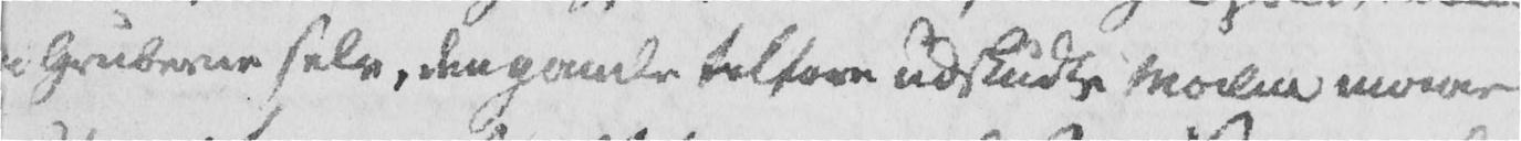i Gruberne selv, den gamle tilforn udskudte Malm maae
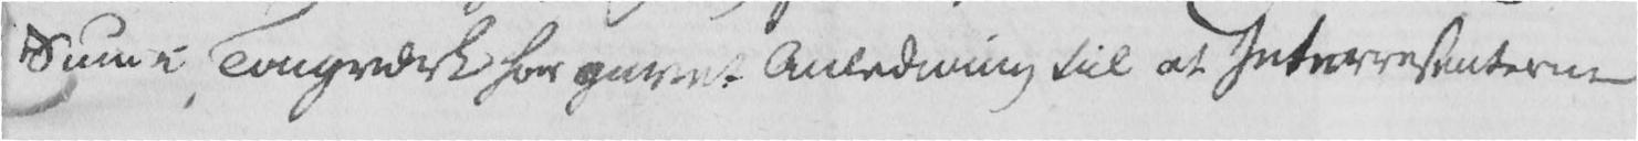Sum i Taugværk har givet Anledning til at Interresenterne
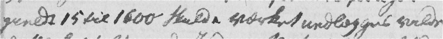gieldt 15 til 1600 Skulde Værket nedlægges vilde
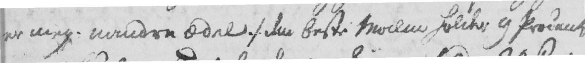er meg. mindre ædel /: den beste Malm holder 9 Procent
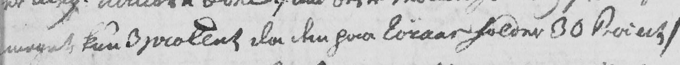meget kun 3 Procent da den paa Røraas holder 30 Procent /
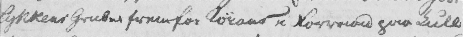Lykkens Gruber fremfor Røraas i Forraad paa Kull
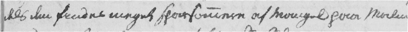dels den findes meget sparsomere af Mangel paa Malm
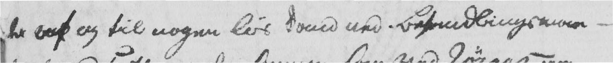det af og til nogen løs Sand ned. Behandlingsmaa-
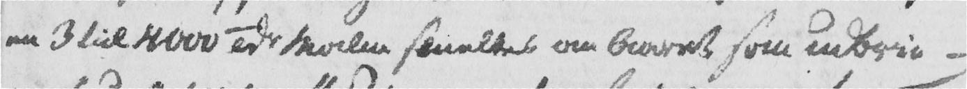en 3 til 4000 Tdr Malm smeltes om Aaret som indbrin-
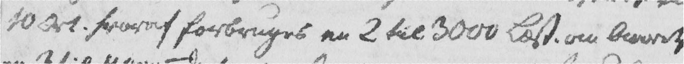10 Ort. hvoraf forbruges en 2 til 3000 Læst. om Aaret
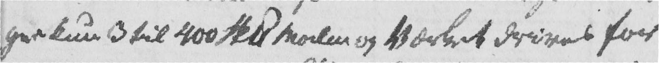ger kun 3 til 400 Skip Malm og Værket drives for
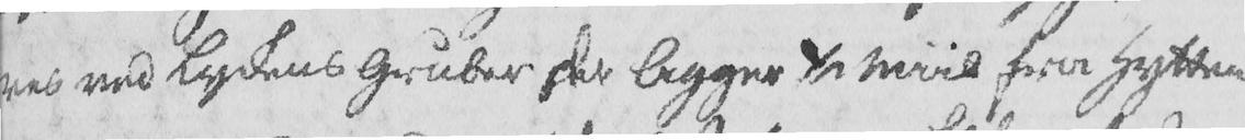ves ved Lyckens Gruber her ligger 1 Miil fra Hytten
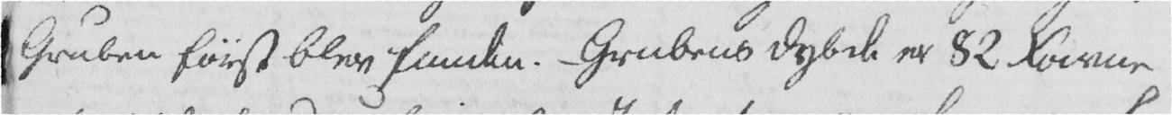Gruben først blev funden. Grubens dybde er 82 Favne
